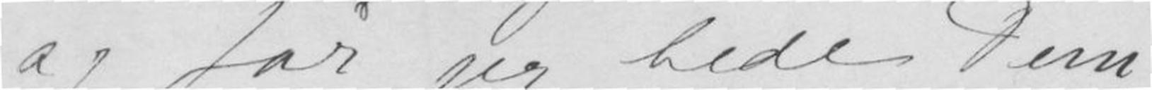og får jeg bede Dem
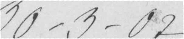30-3-07.
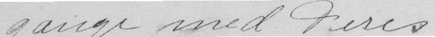gange med Deres
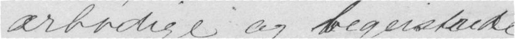ærbødige og begeistræde
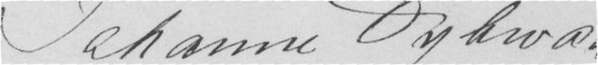Johanna Dybwad
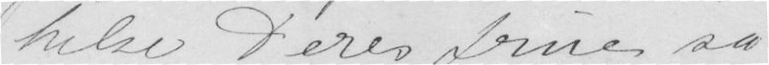hilse Deres frue så
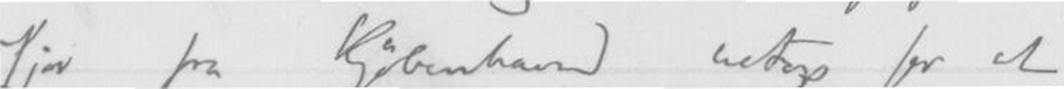jeg ifjor fra Kjøbenhavn netop for at
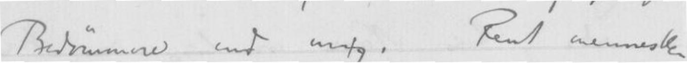Bedømmere end mig. Rent menneske-
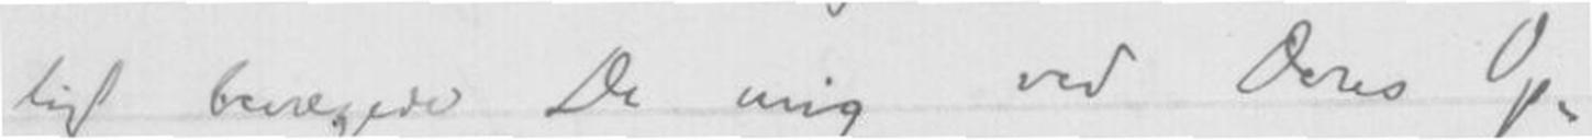ligt bevegede De mig ved Deres Op-
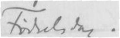Fødselsdag.
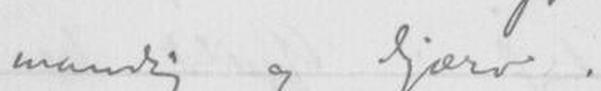mandig og Gjærv.
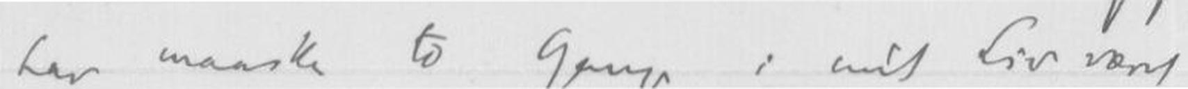jeg har maaske to Gange i mit Liv været
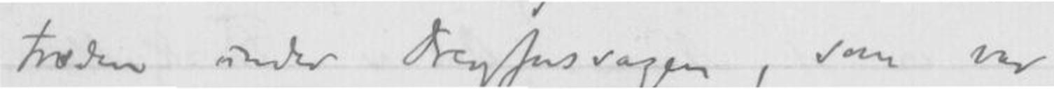træden under Dreyfussagen, som var
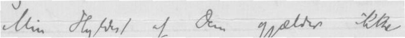Min Hyldest af Dem gjælder ikke
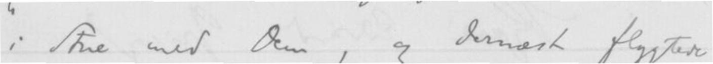i Stue med Dem, og dernæst flygtede
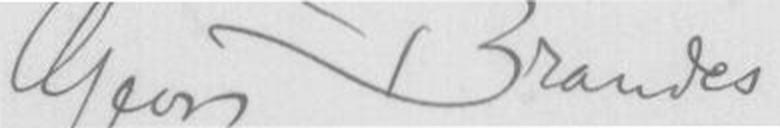Georg Brandes
